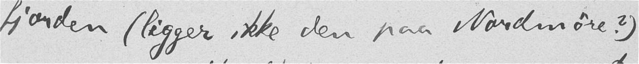fjorden (ligger ikke den paa Nordmøre?)
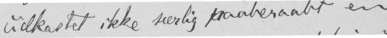udkastet ikke særlig paaberaabt en
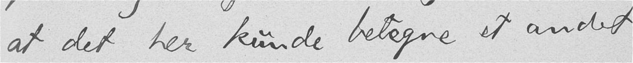at det her kunde betegne et andet
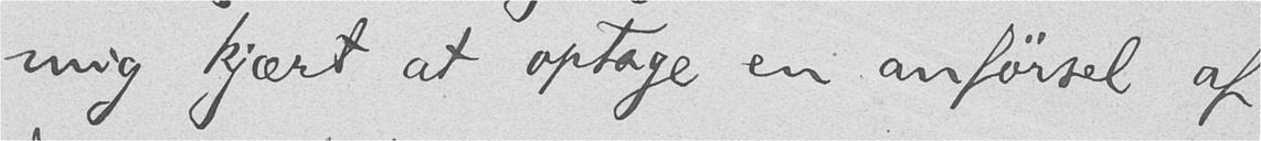mig kjært at optage en anførsel af
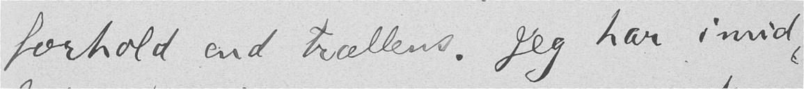forhold end trællens. Jeg har imid-
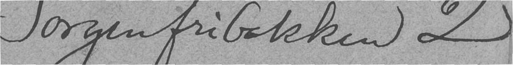Sorgenfribakken 2
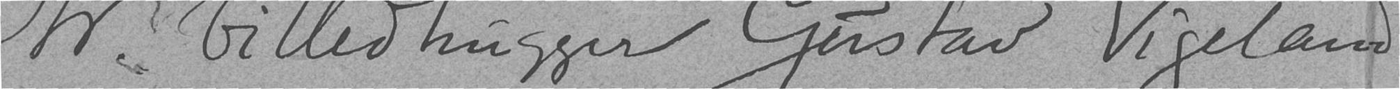Hr. billedhugger Gustav Vigeland
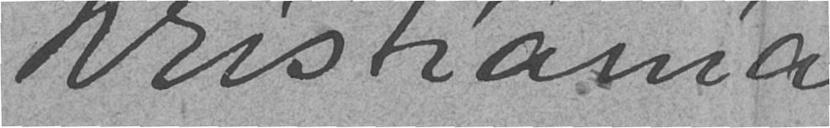Kristiania
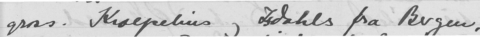gross. Kroepelins og Isdahls fra Bergen,
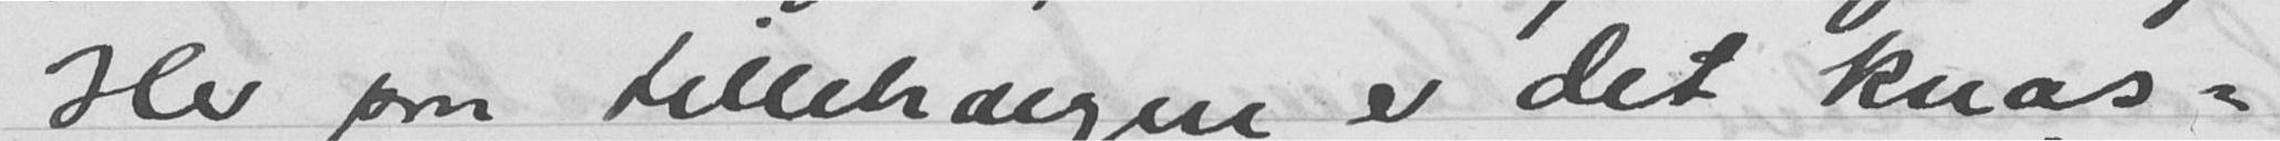Her paa Lillehaugen er det knas-
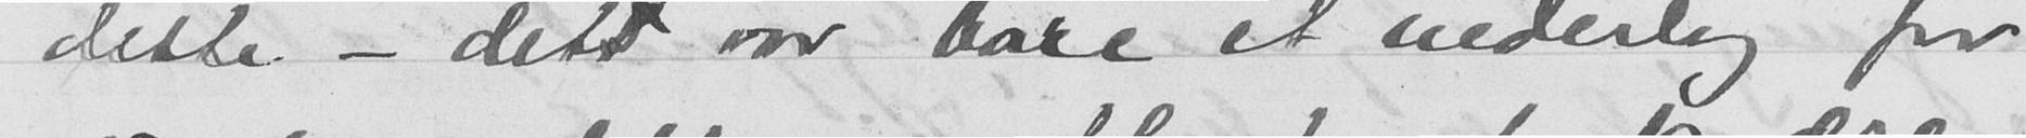dette - dett var bare et nederlag for
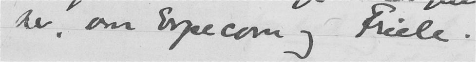her. von Erpecom og Friele.
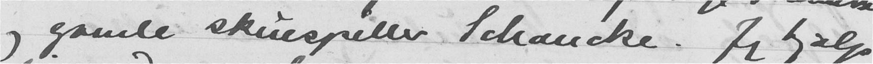og gamle skuespiller Schancke. Jeg hjalp
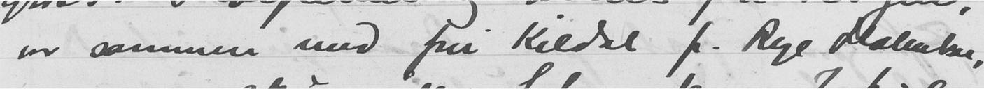var sammen med fru Kildal fr. Rye Holmboe,
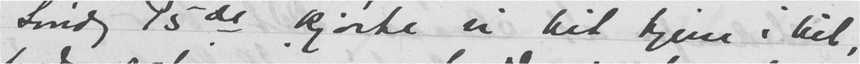Lørdag 15de kjørte vi hit hjem i bil,
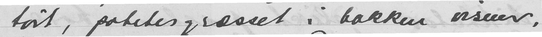tørt, potetesgræsset i bakken visner,
